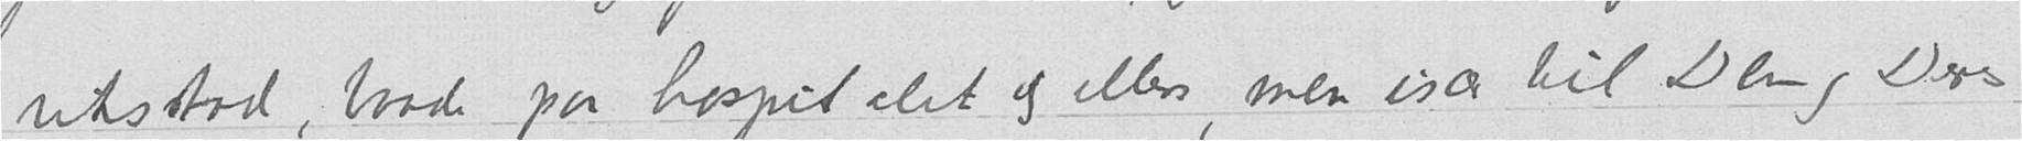riksstad, baade paa hospitalet og ellers, men især til Dem og Deres
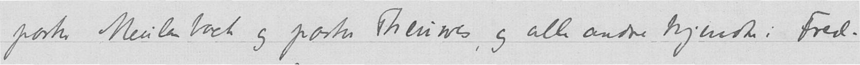pastor Meulenbeck og pastor Theuwes, og alle andre kjendte i Fred-
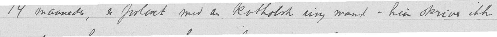14 maaneder, er forlovet med en katholsk ung mand – hun skriver ikke
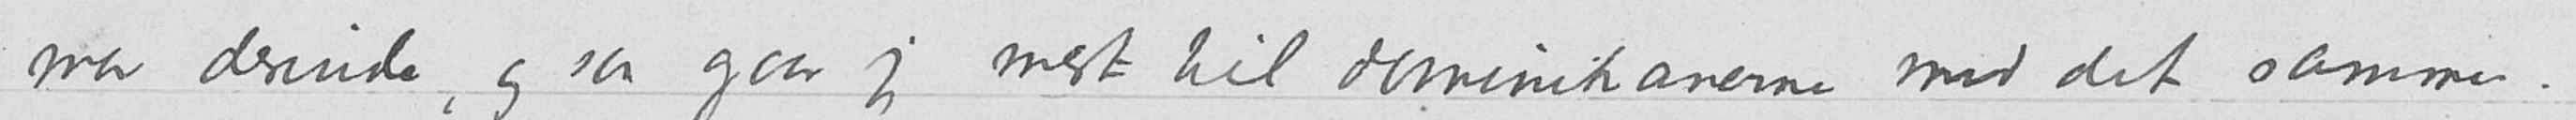mor derinde, og saa gaar jeg mest til dominikanerne med det samme.
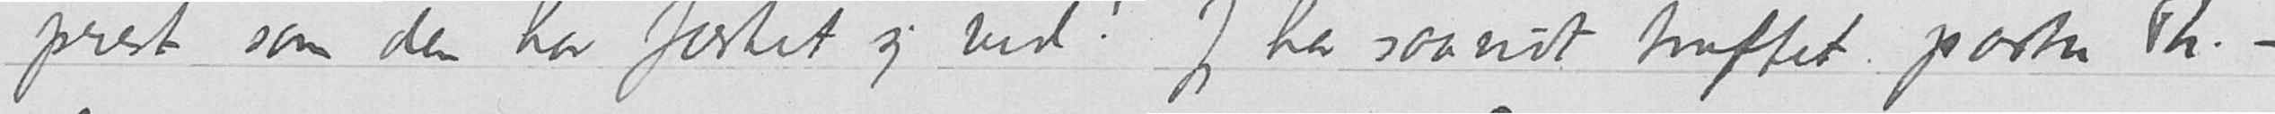prest som den har fæstet sig ved! Jeg har saavidt truffet pastor Th. –
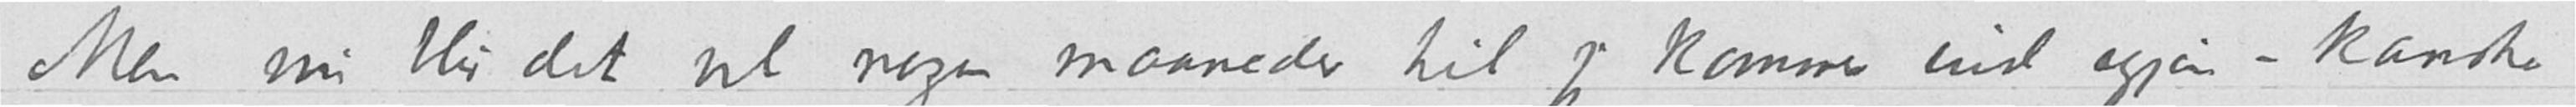Men nu blir det vel nogen maaneder til jeg kommer ind igjen – kanske
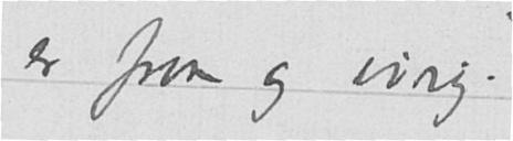er from og ivrig.
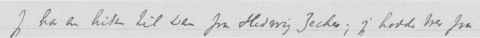Jeg har en hilsen til Dem fra Hedwig Zecher; jeg hadde brev fra
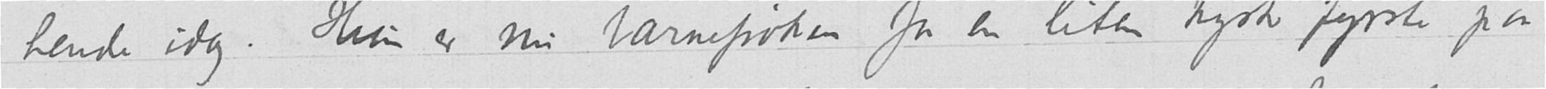hende idag. Hun er nu barnefrøken for en liten tysk fyrste paa
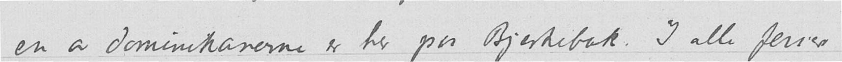en av dominikanerne er her paa Bjerkebæk. I alle ferier
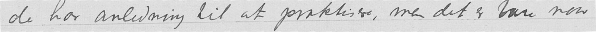de har anledning til at praktisere, men det er bare naar
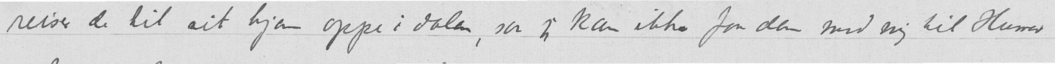reiser de til sit hjem oppe i dalen, saa jeg kan ikke faa dem med mig til Hamar
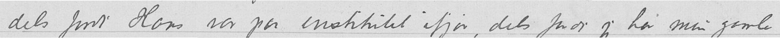dels fordi Hans var paa institutet ifjor, dels fordi jeg har min gamle
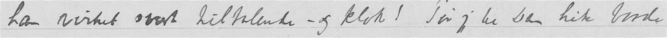han virket svært tiltalende – og klok! Tør jeg be Dem hilse baade
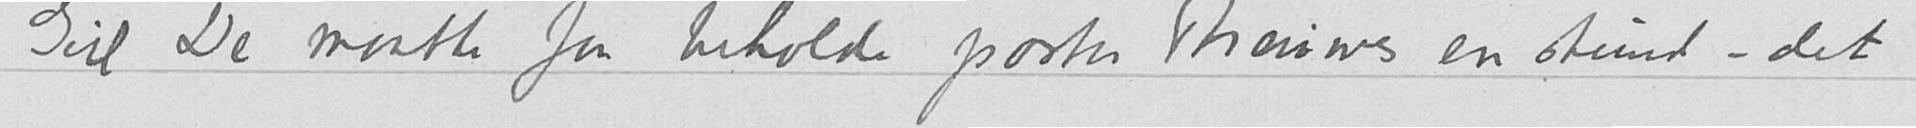Gid De maatte faa beholde pastor Theeuwes en stund – det
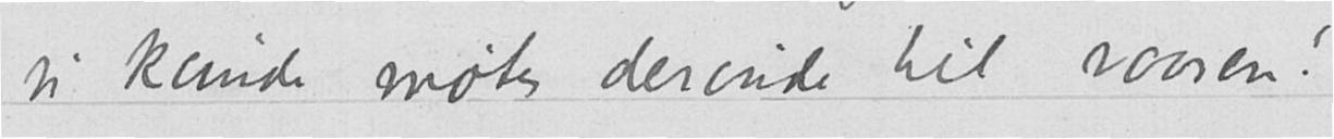vi kunde møtes derinde til vaaren!
